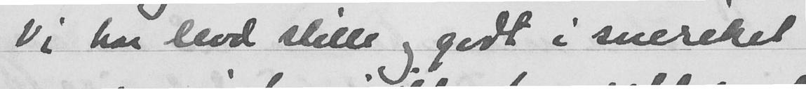Vi har levd stille og godt i sneriket
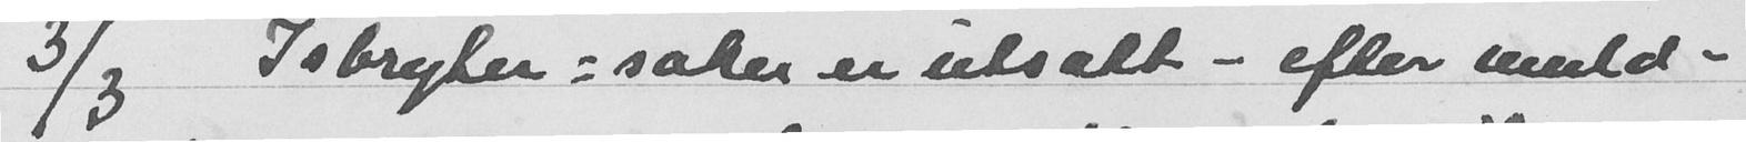3/3 Isbryter-saken er utsatt - efter muld-
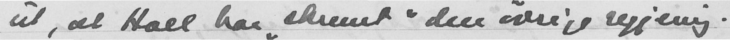ut, at Hoel har "skremt" den øvrige regjering.
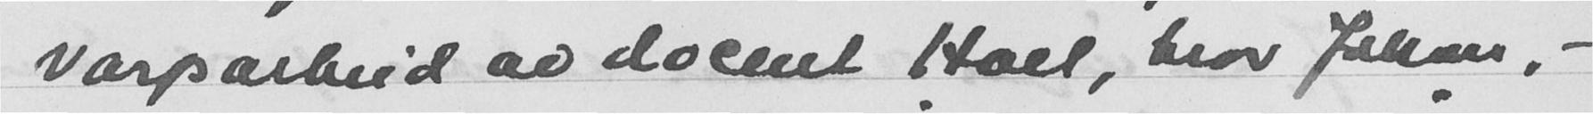varparbeid av docent Hoel, tror Johan, -
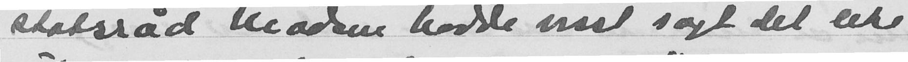statsråd Madsen hadde visst sagt det like
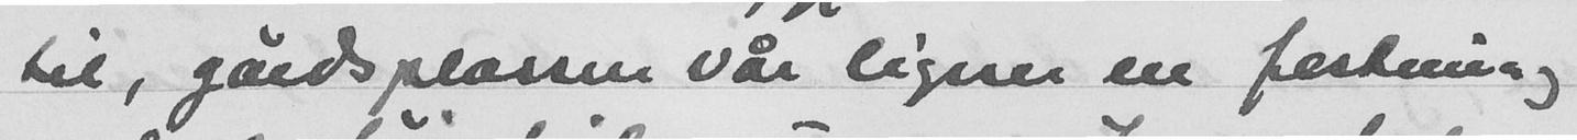til, gårdsplassen vår ligner en festning
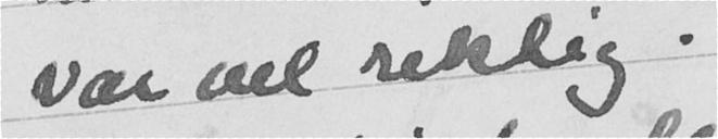var vel riktig.
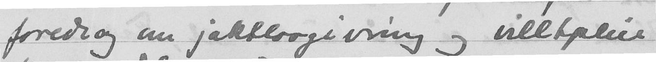foredrag om jaktlovgivning og villtpleie
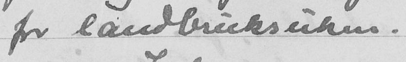for landbruksuken.
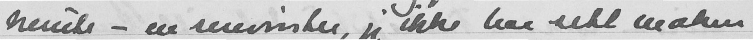herute - en snevinter, jeg ikke har sett maken
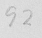92
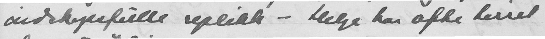ondskapsfulle replikk - Helge har ofte tirret
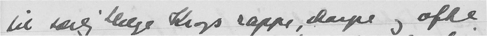til særlig Helge Krogs rappe, skarpe og ofte
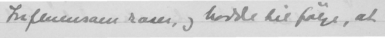Fra flemmesam raser, og hadde til følge, at
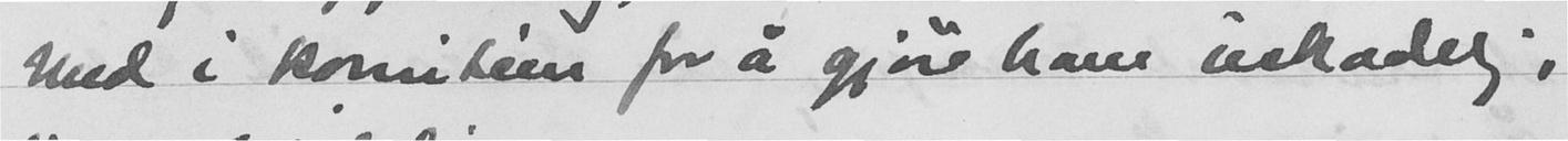med i komitéen for å gjøre ham uskadelig,
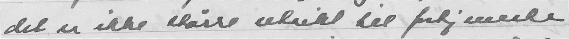det er ikke større utsikt til fortjeneste
